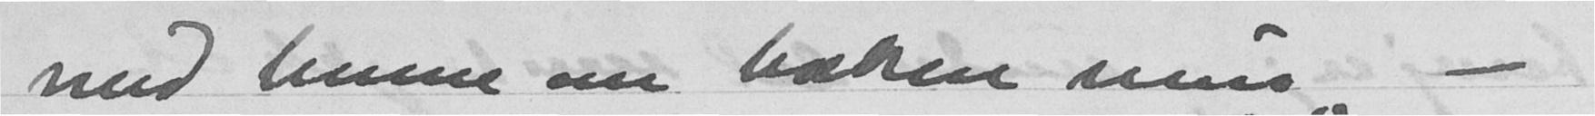med henne om boken min. -
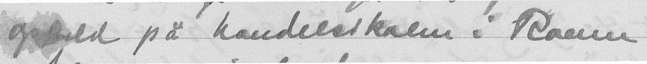ophold på handelsskolen i Hauen
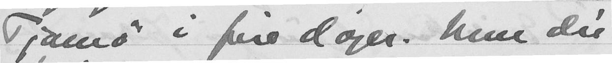Tromsø i fire dager. Men dèr
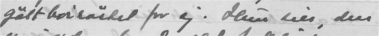gått høirøstet for sig. Hun sier, den
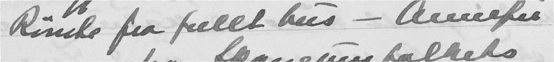Rømte fra fullt hus - Annefri,
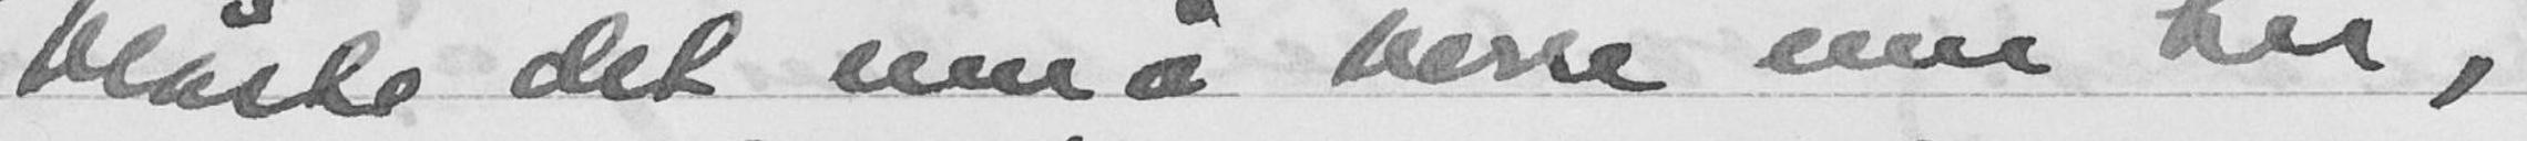blåste det ennå verre enn her,
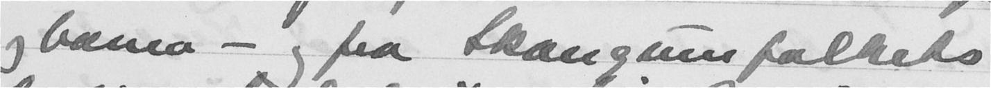og barna - og fra Skaugumsfolkets
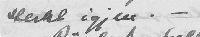sterkt igjen. -
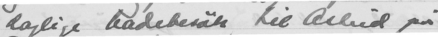daglige badebesøk til Astrid på
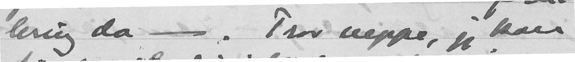lering da -. Tror neppe, jeg kan
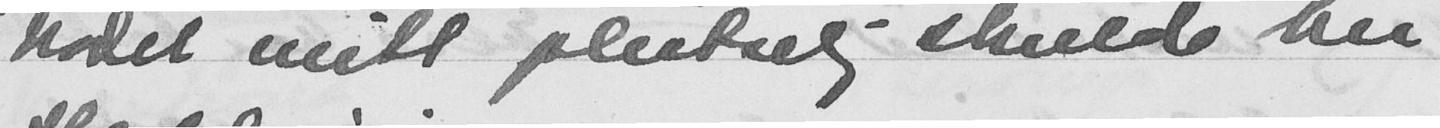hodet mitt plutselig skulde bli
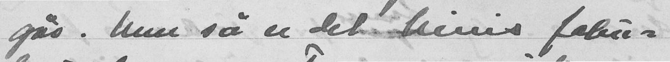går. Men så er det his fabu-
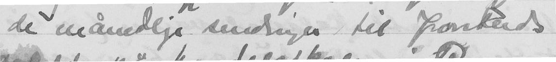de månedlige sendinger til Jonteeds
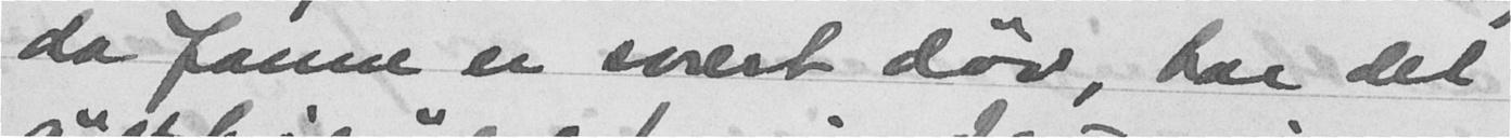da Janne er svert døv, har det
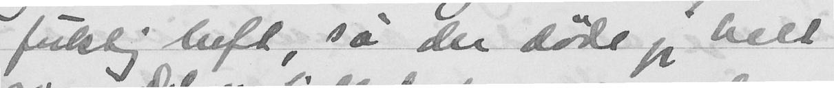fuktig luft, så der døde jeg helt
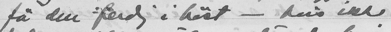få den ferdig i høst - om ikke
